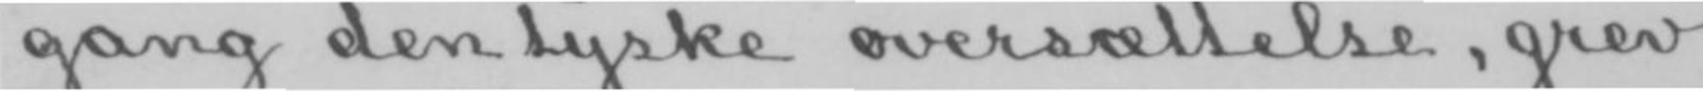gang den tyske oversættelse, grev
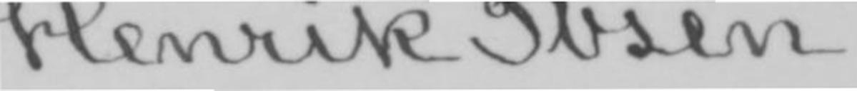Henrik Ibsen.
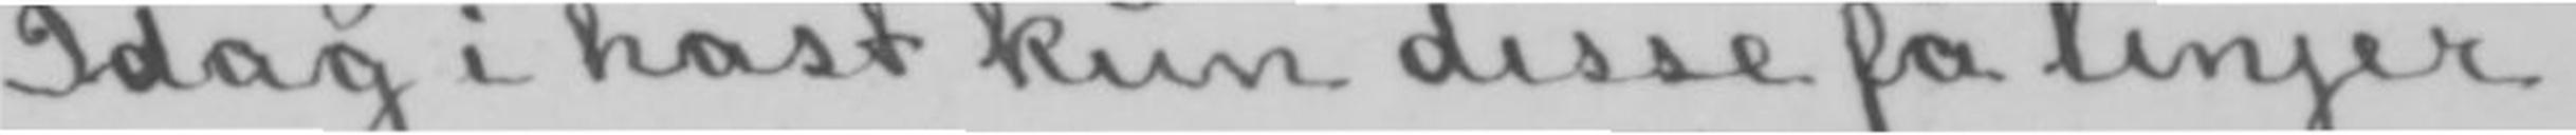Idag i hast kun disse få linjer
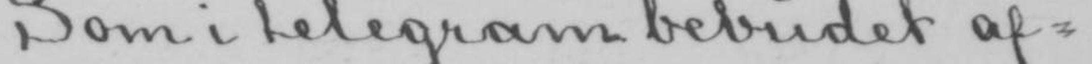Som i telegram bebudet af-
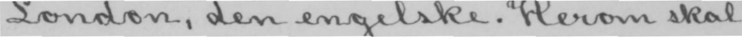London, den engelske. Herom skal
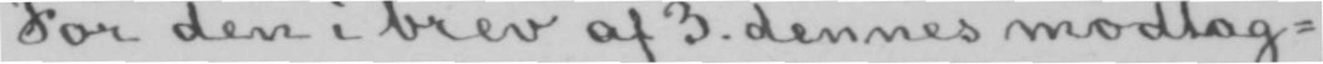For den i brev af 3. dennes modtag-
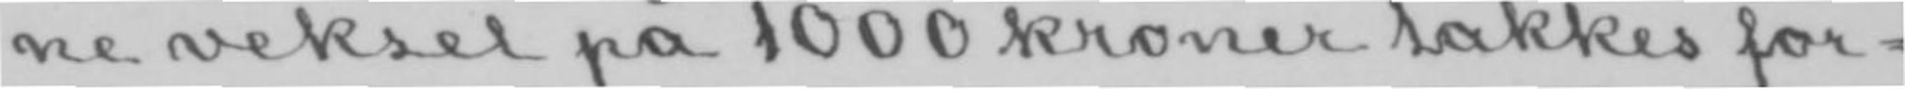ne veksel på 1000 kroner takkes for-
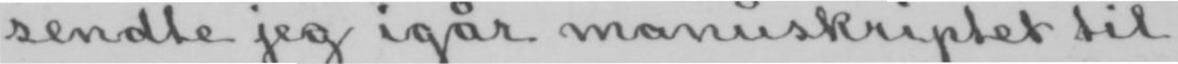sendte jeg igår manuskriptet til
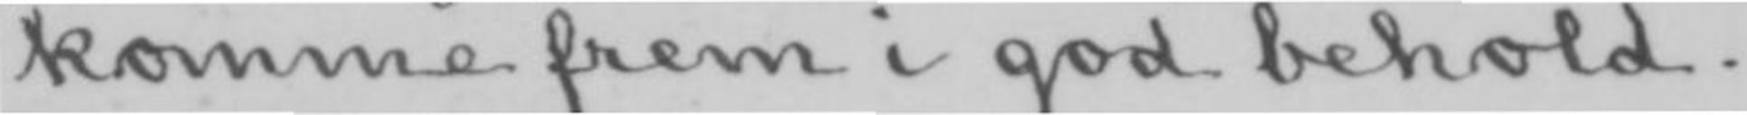komme frem i god behold.
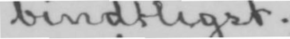bindtligst.
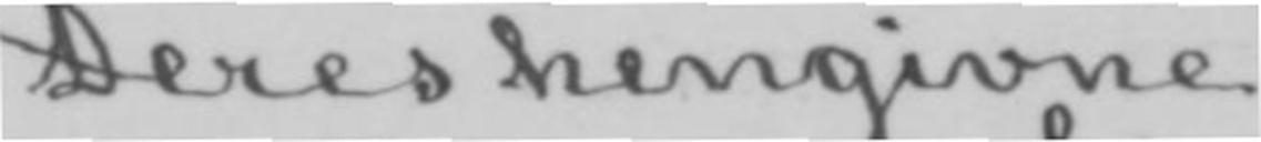Deres hengivne
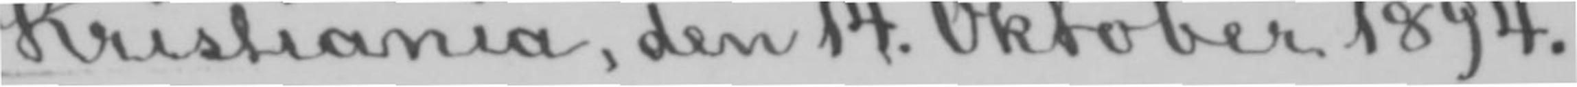Kristiania, den 14. Oktober 1894.
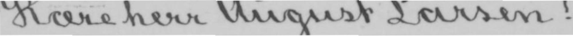Kære herr August Larsen!
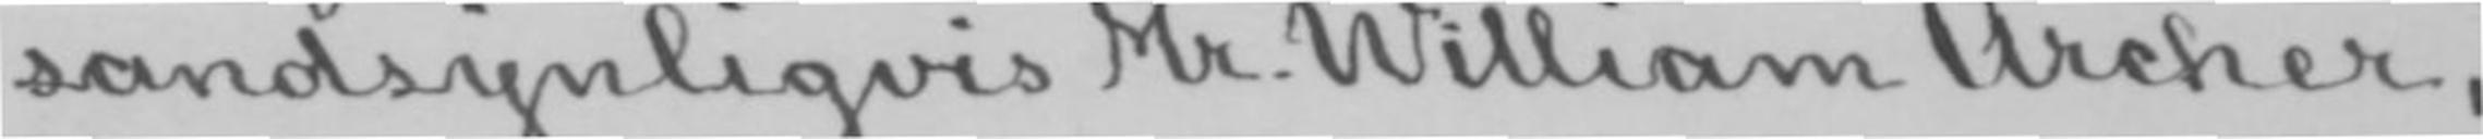sandsynligvis Mr. William Archer,
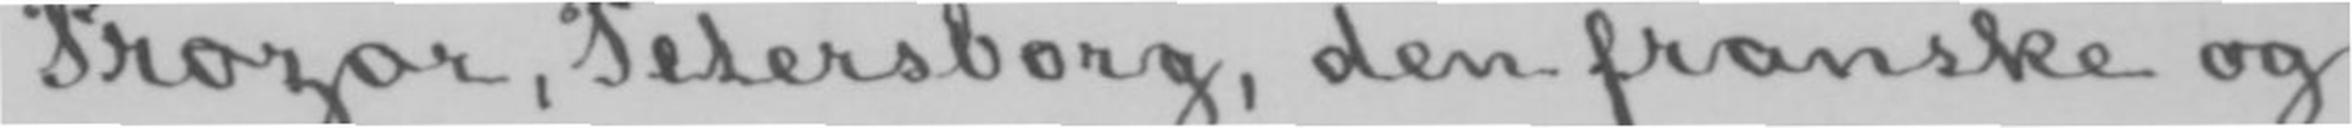Prozor, Petersborg, den franske og
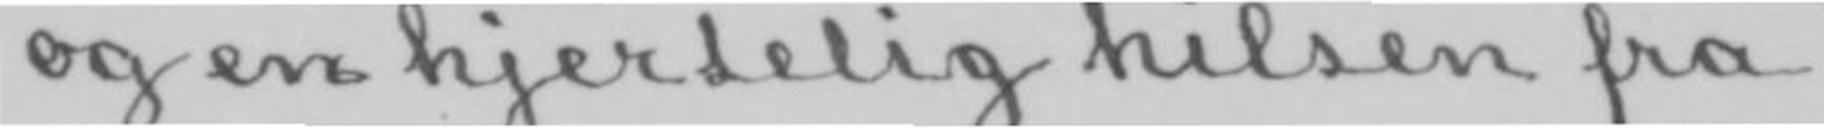og en hjertelig hilsen fra
